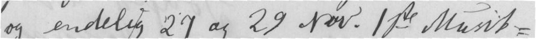og endelig 27 og 29 Nov. 1ste Musik-
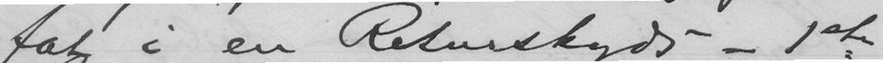fat i en Returskyds - 1ste
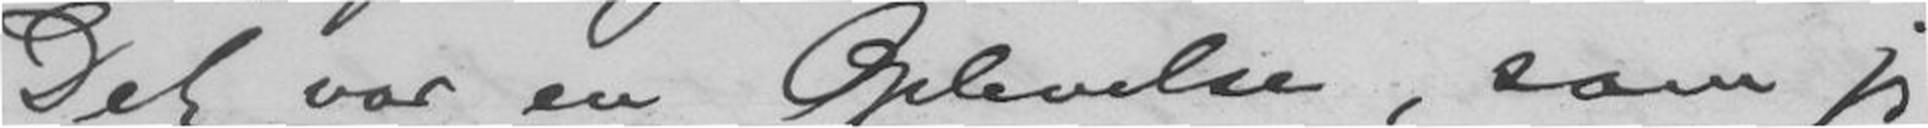Det var en Oplevelse, som jeg
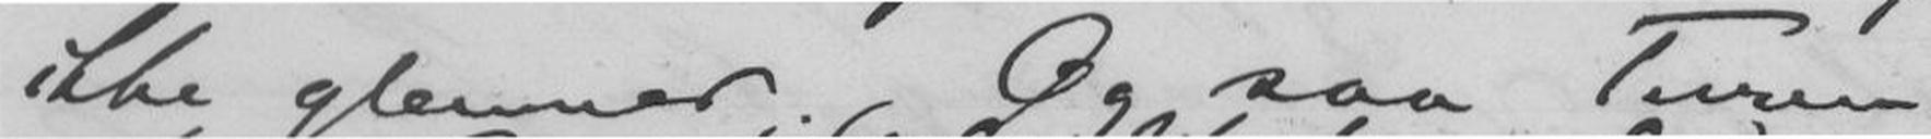ikke glemmer. Og saa Turen
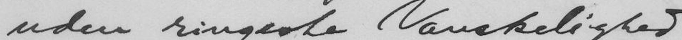uden ringeste Vanskelighed
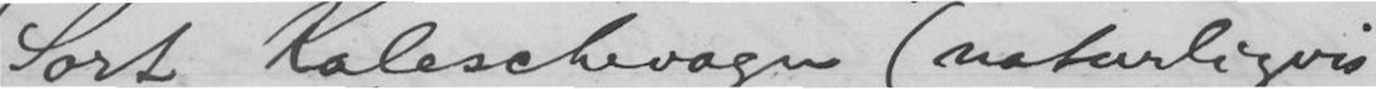Sort Kaleschevogn (naturligvis
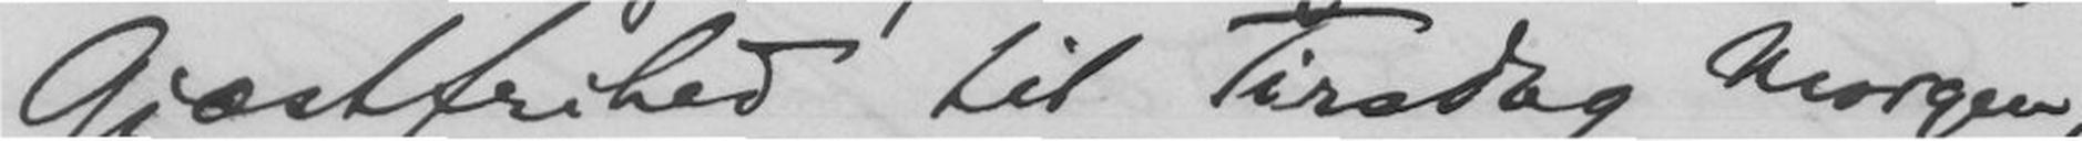Gjæstfrihed til Tirsdag Morgen,
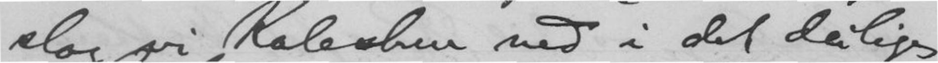slog vi Kalehen ned i det deilige
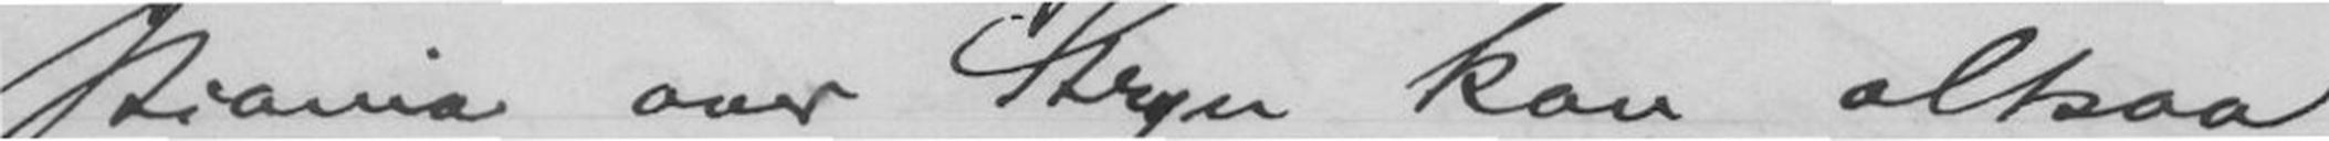stiania over Stryn kan altsaa
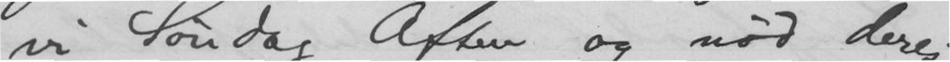vi Søndag Aften og nød deres
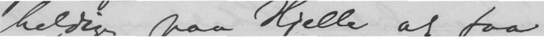heldige paa Hjelle at faa
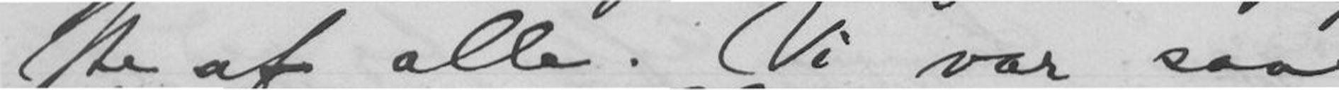ste af alle. Vi var saa
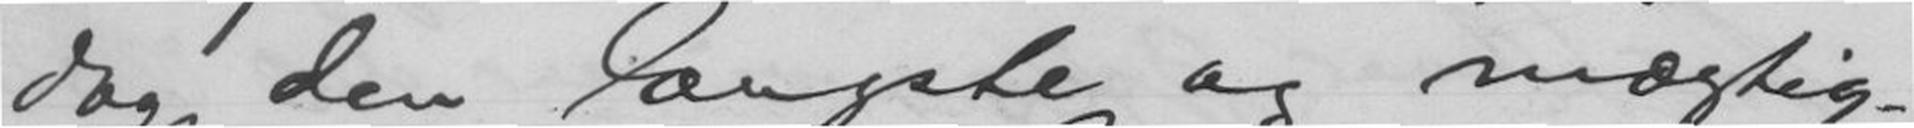dog den længste og mægtig-
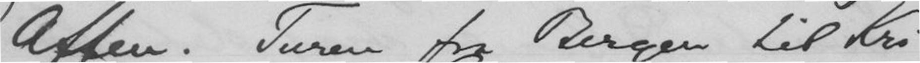Aften. Turen fra Bergen til Kri-
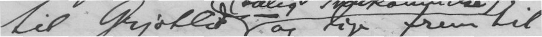til Grjotlid (salig Ihukommelse) og lige frem
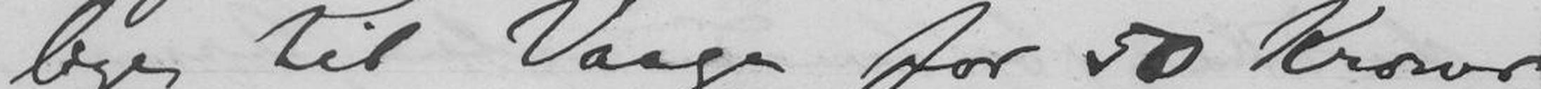lige til Vaage for 50 Kroner.
